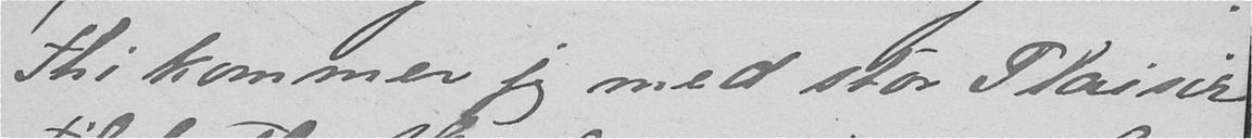Thi kommer jeg med stor Plaisir
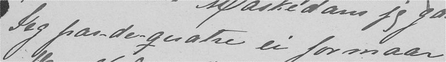Jeg pas-de-quatre ei formaar
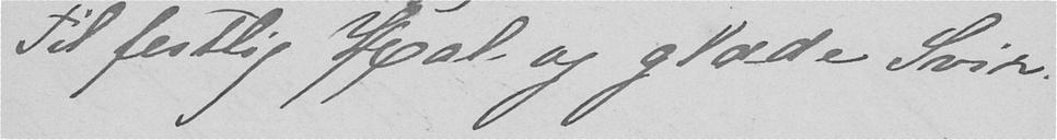Til festlig Hal og glæde Svir.
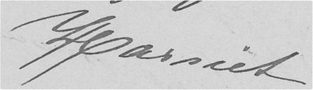Harriet
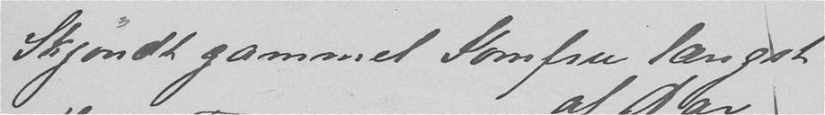Skjøndt gammel Jomfru længst
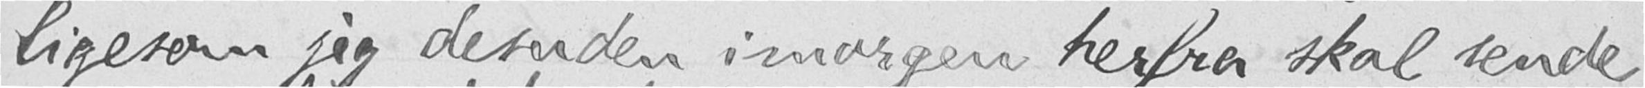ligesom jeg desuden imorgen herfra skal sende
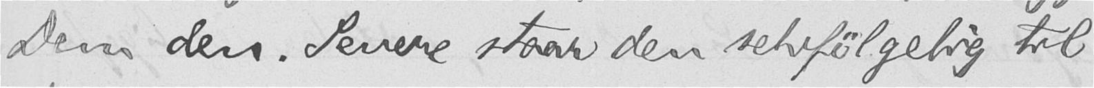Dem den. Senere staar den selvfølgelig til
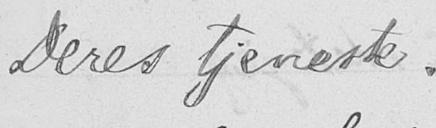Deres tjeneste.
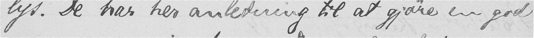lys. De har her anledning til at gjøre en god
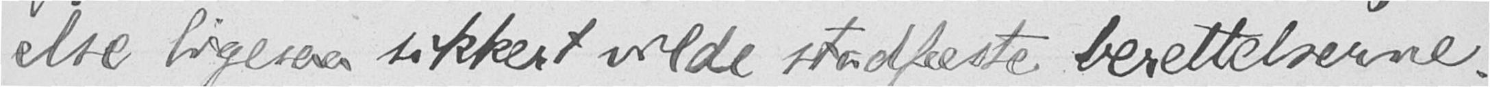else ligesaa sikkert vilde stadfæste berettelserne.
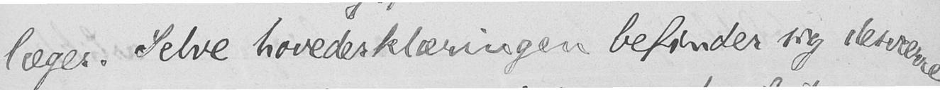læger. Selve hovederklæringen befinder sig desværre
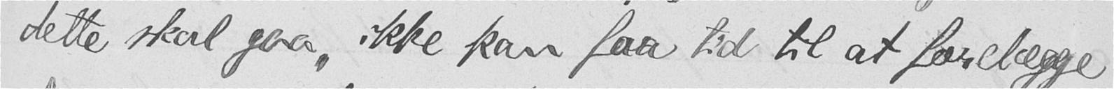dette skal gaa, ikke kan faa tid til at forelægge
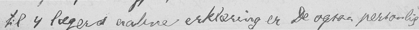til 4 lægers aabne erklæring er De ogsaa personlig
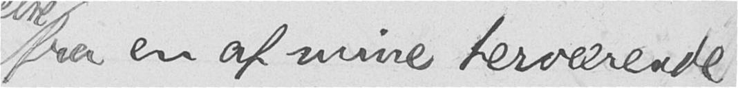fra en af mine herværende
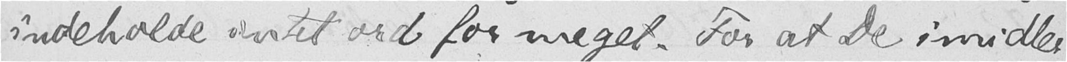indeholde intet ord for meget. For at De imidler-
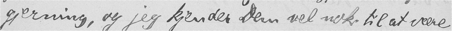gjerning, og jeg kjender Dem vel nok til at være
